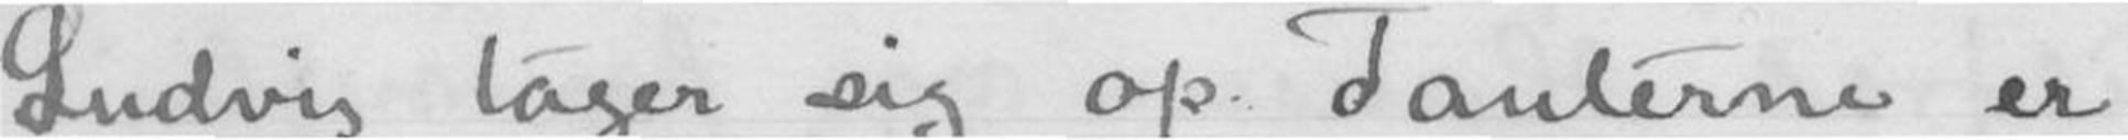Ludvig lager sig op. Tanterne er
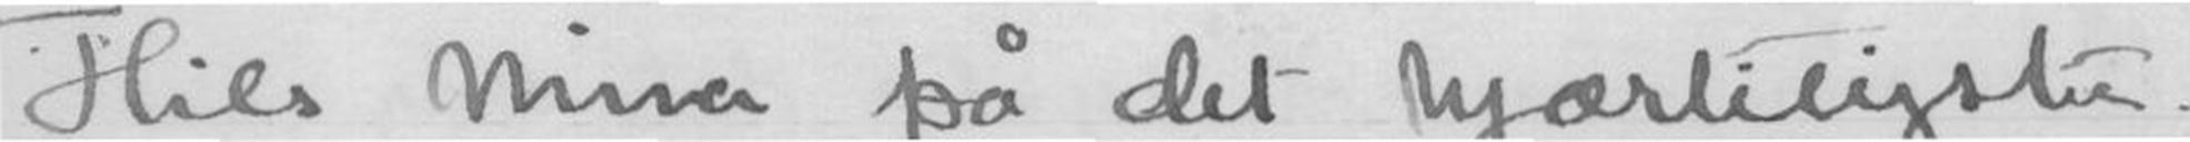Hils Nina på det hjærteligste
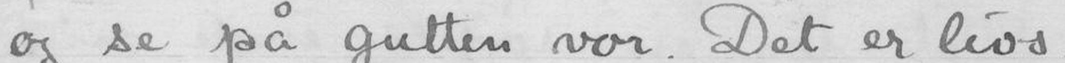og se på gutten vor. Det er livs-
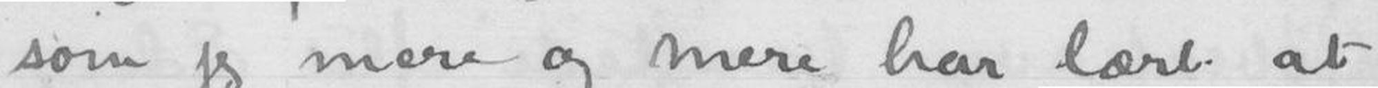som jeg mere og mere har lært at
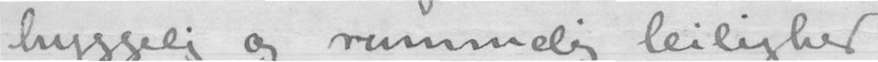hyggelig og rummelig leilighed.
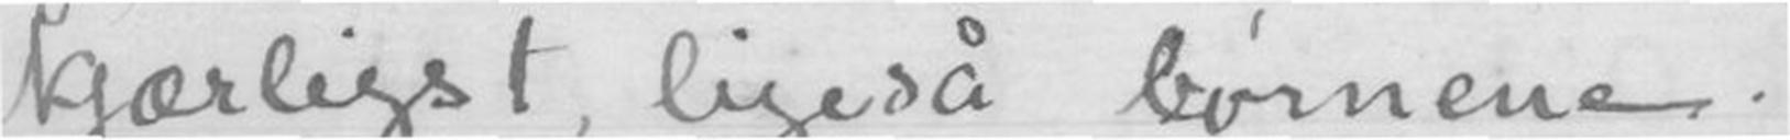kjærligst, ligeså børnene.
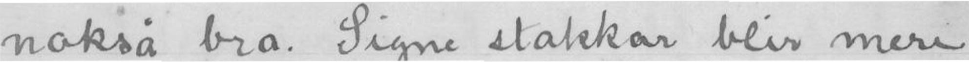nokså bra. Signe stakkar blir mere
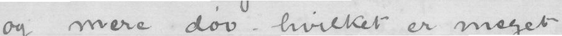og mere døv hvilket er meget
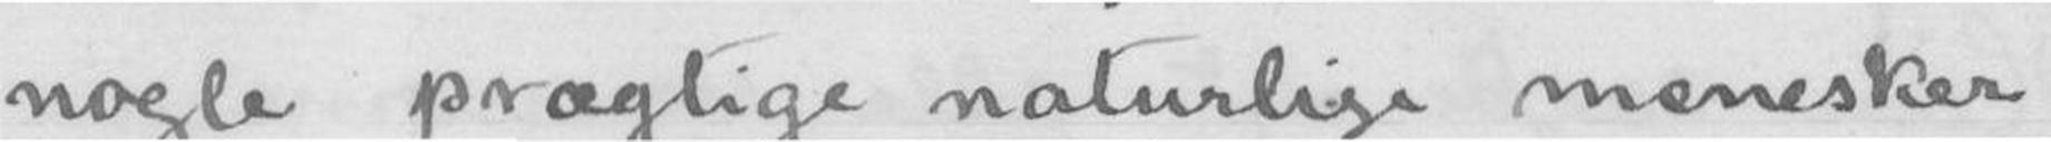nogle prægtige naturlige menesker
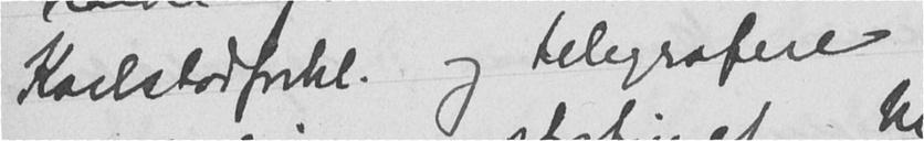Karlstadforhl. og telegrafere
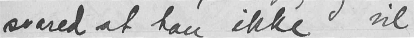svared at han ikke vil
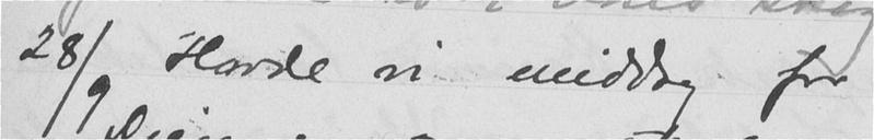28/9 Havde vi middag for
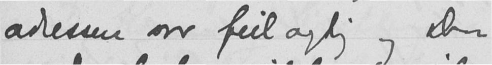adressen var feilagtig og Don
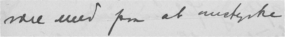være med paa at omstyrke
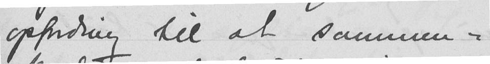opfording til at sammen-
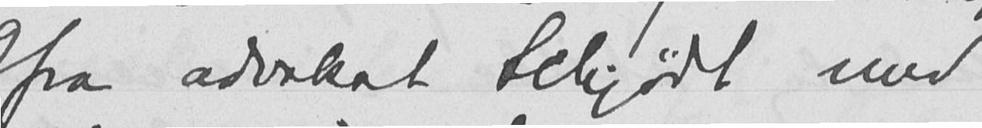fra advokat Schjødt med
